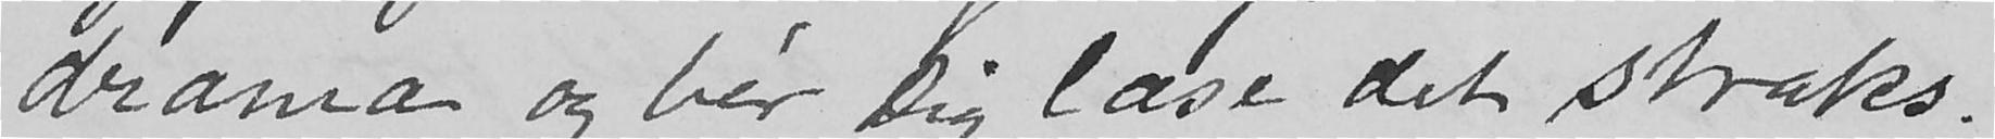drama og ber dig læse det straks.
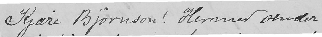Kjære Bjørnson! Hermed sender
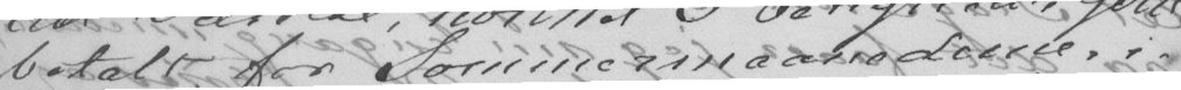betalt for Sommermaanederne i-
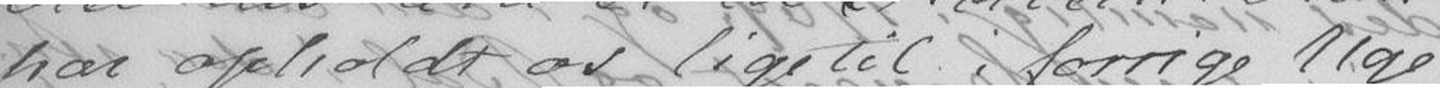har opholdt os ligetil forrige Uge,
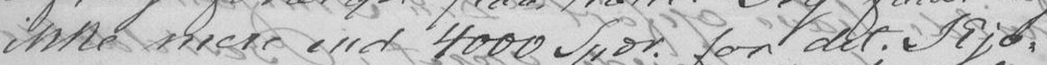ikke mere end 4000 Spdo. for de. Kjø-
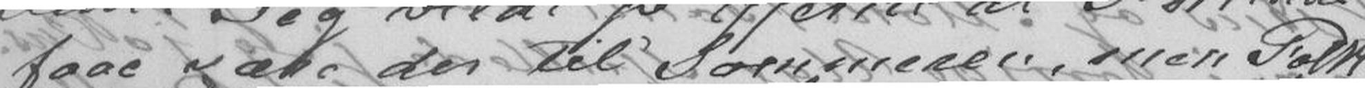faae være der til Sommeren, men Folk
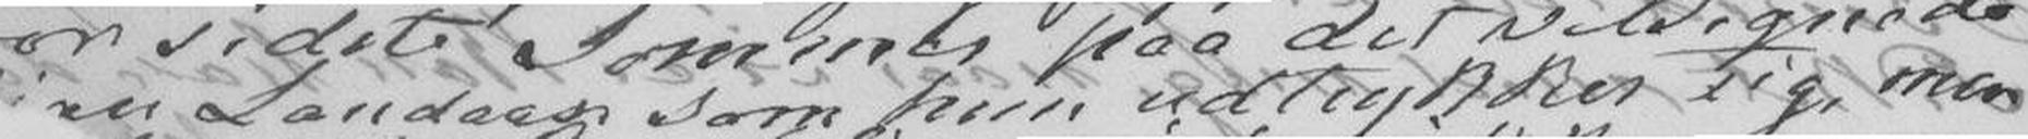an Landaas som hun udtrykker sig, men
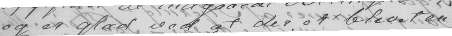og er glad ved at der er blevet en
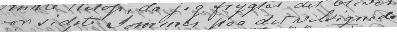or sidste Sommer paa det velsignede
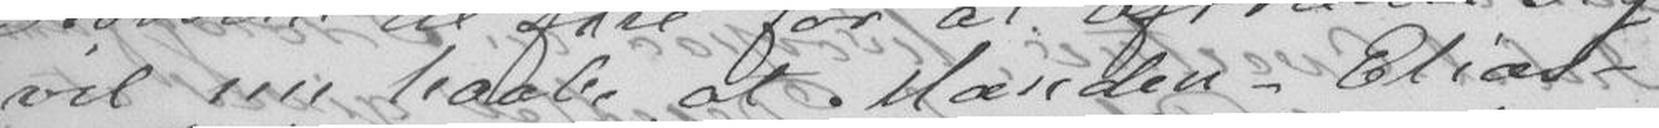vil nu haabe at Manden - Elias -
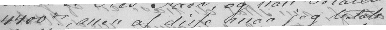4400do, men af disse maa jeg betale
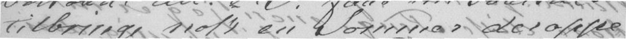tilbringe nok en Sommer deroppe
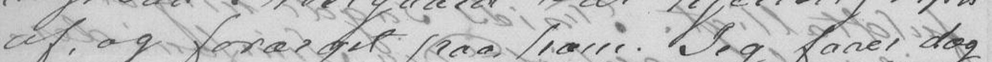af, og forarget paa ham. Jeg faaer dog
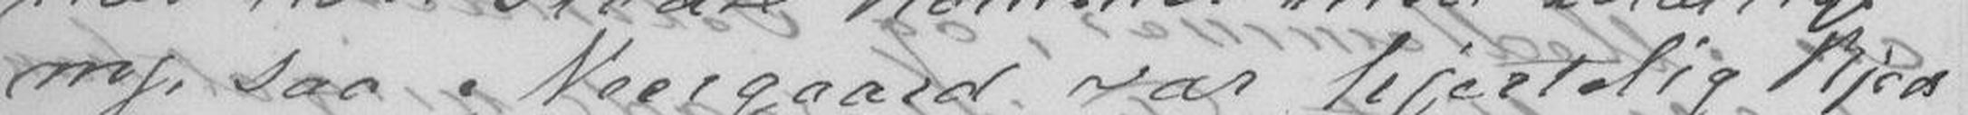ny, saa Neergaard var hjertelig kjed
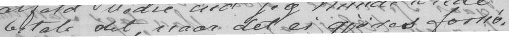betale det, naar det ei gjøres fornø-
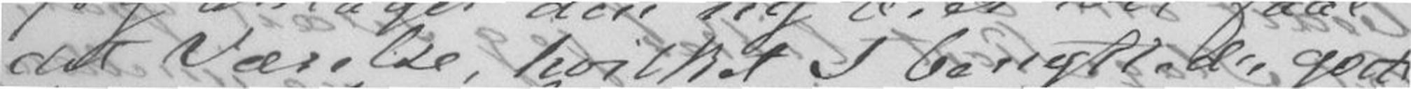det Værelse, hvilket I benyttedes godt
